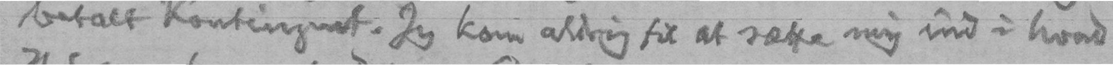betalt Kontingent. Jeg kom aldrig til at sætte mig ind i hvad
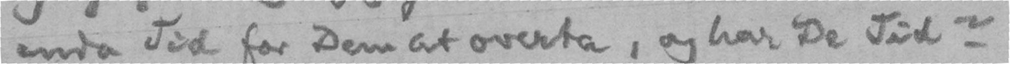enda Tid for Dem at overta, og har De Tid?
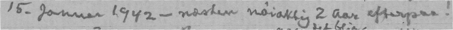15. Januar 1942 - næsten nøiaktig 2 Aar efterpaa!
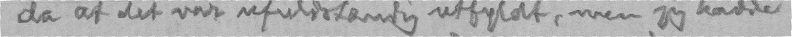da at det var ufuldstændig utfyldt, men jeg hadde
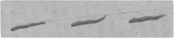- - -
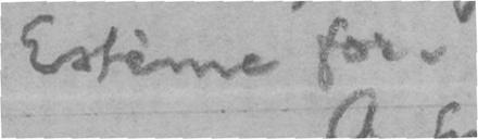Estème for.
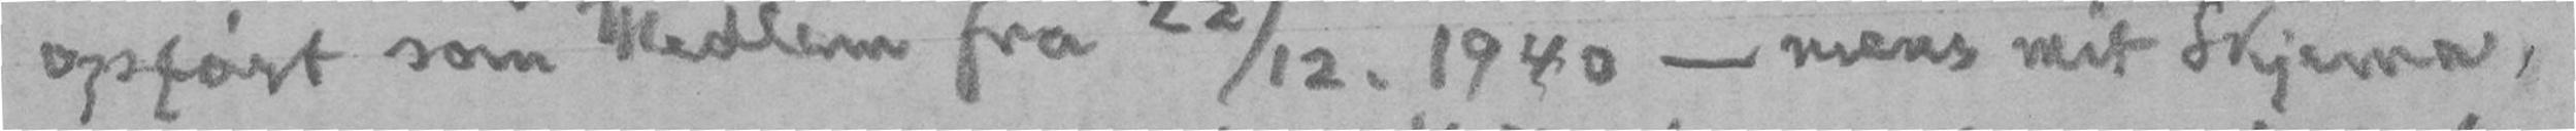opført som Medlem fra 22/12. 1940 - mens mit Skjema,
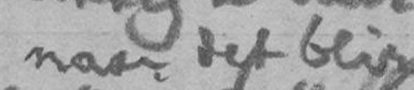naar det blir
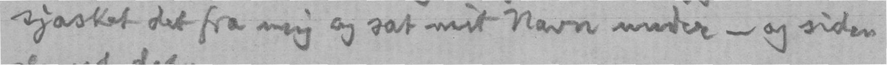sjasket det fra mig og sat mit Navn under - og siden
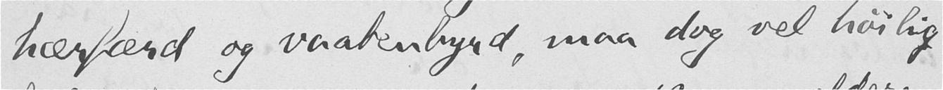hærfærd og vaabenbyrd, maa dog vel høilig
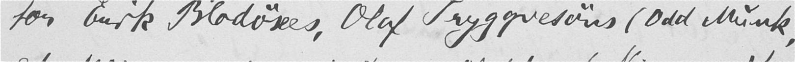for Erik Blodøxes, Olaf Tryggvesøns (Odd Munk,
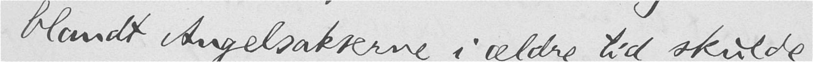blant Angelsakserne i ældre tid skulde
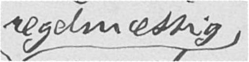regelmæssig
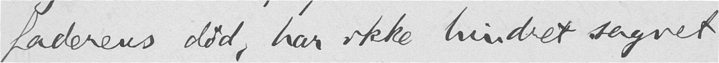faderens død, har ikke hindret sagnet
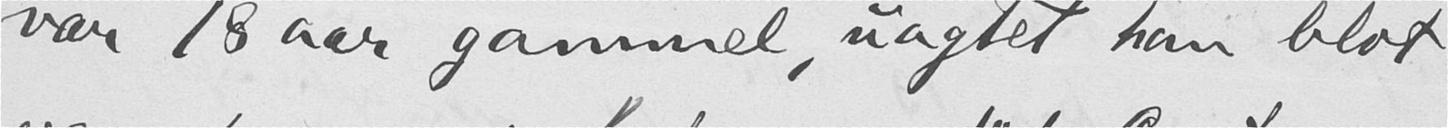var 18 aar gammel, uagtet han blot
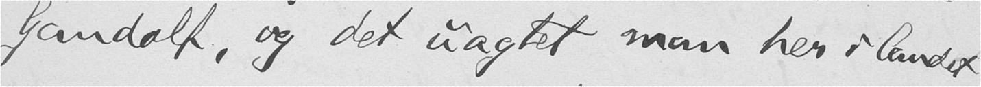Gandalf, og det uagtet man her i landet
